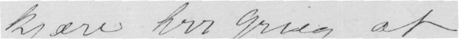kjære hrr Grieg at
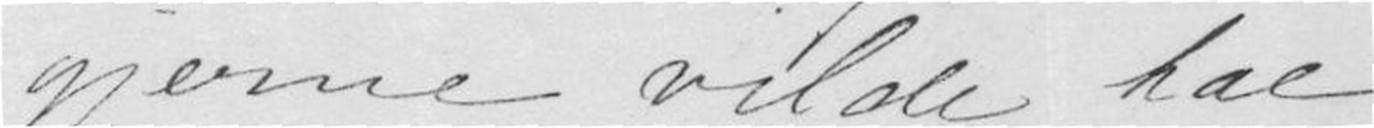gjerne vilde hae
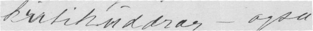kritikuddrag - også
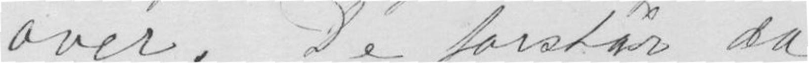over. De forstår da
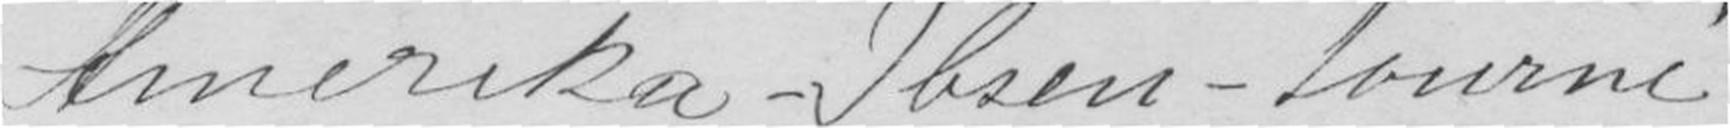Amerika-Ibsen-tourné
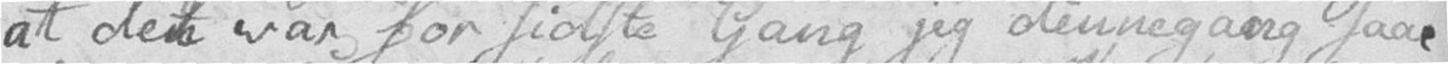at det var for sidste Gang jeg dennegang saae
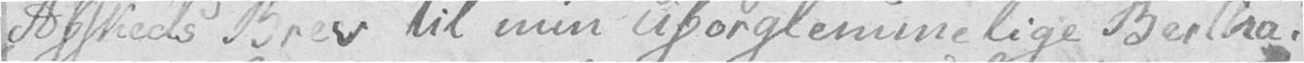Afskeds Brev til min uforglemmelige Bertha!
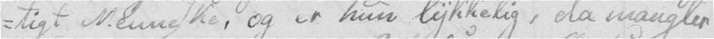-tigt Menneske, og er hun lykkelig, da mangler
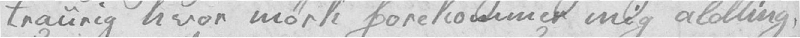traurig hvor mørk forekommer mig aldting,
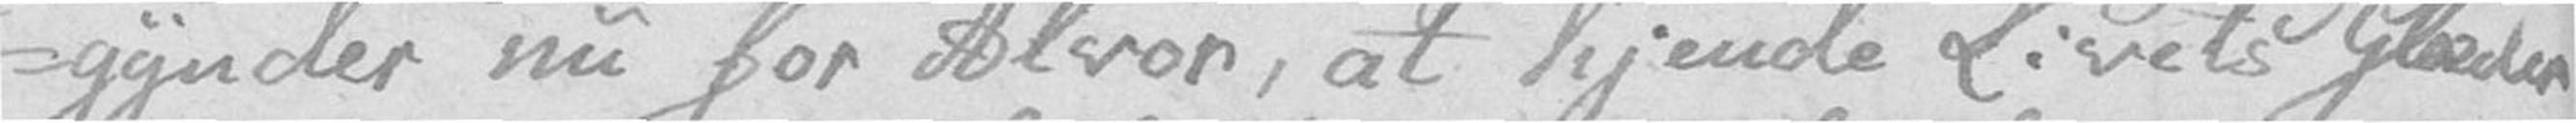-gynder nu for Alvor, at kjende Livets Glæder
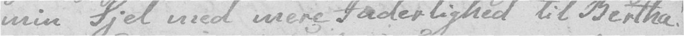min Sjel med mere Inderlighed til Bertha!
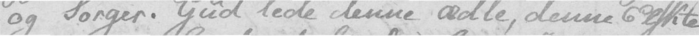og Sorger. Gud lede denne ædle, denne Elskte
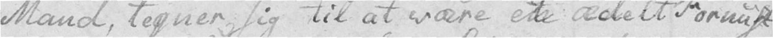Mand, tegner sig til at være et ædelt Fornuf
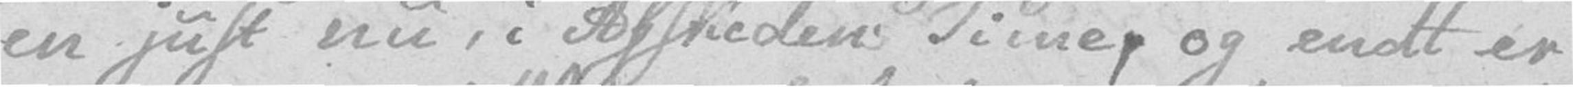en just nu, i Afskedens Time, og endt er
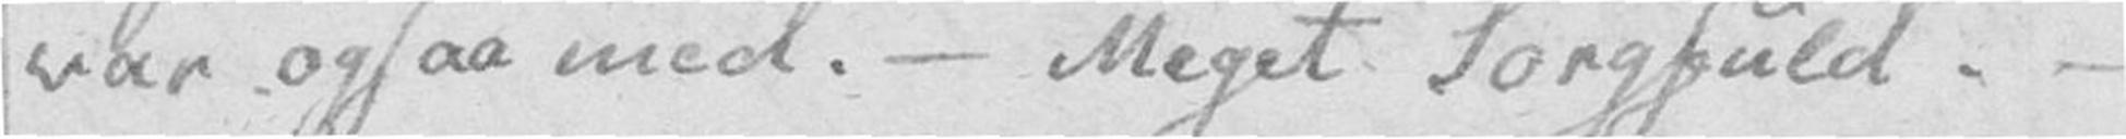var ogsaa med. – Meget Sorgfuld. –
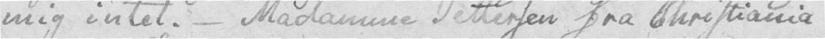mig intet. – Madamme Pettersen fra Christiania
